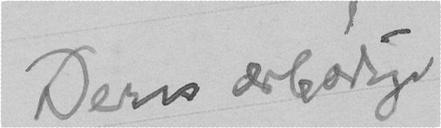Deres ærbødige
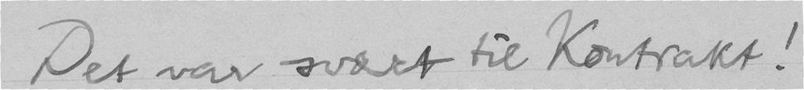Det var svært til Kontrakt!
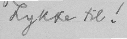Lykke til!
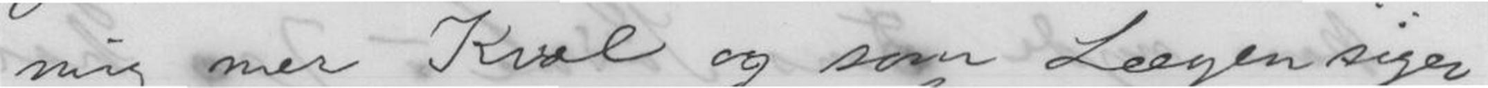mig mer Kval og som Lægen siger
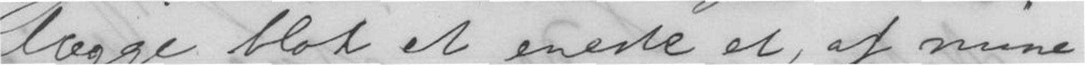lægge blot et eneste et, af mine
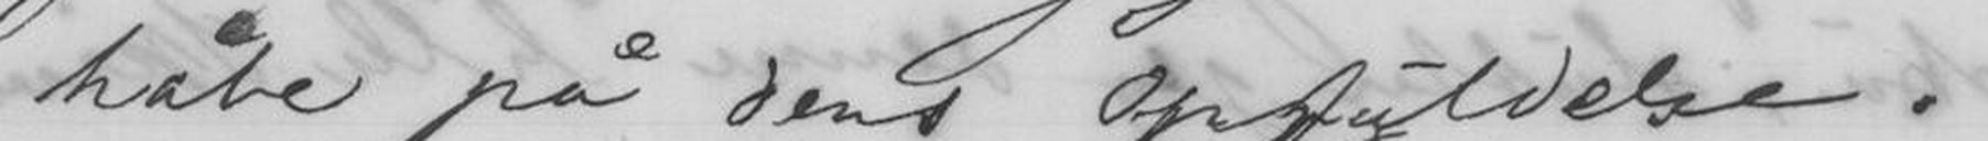håbe på dens Opfyldelse.
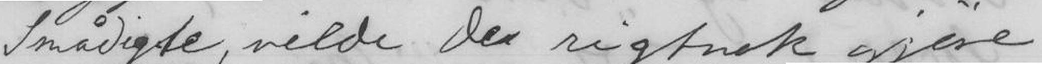Smådigte, vilde De rigtnok gjøre
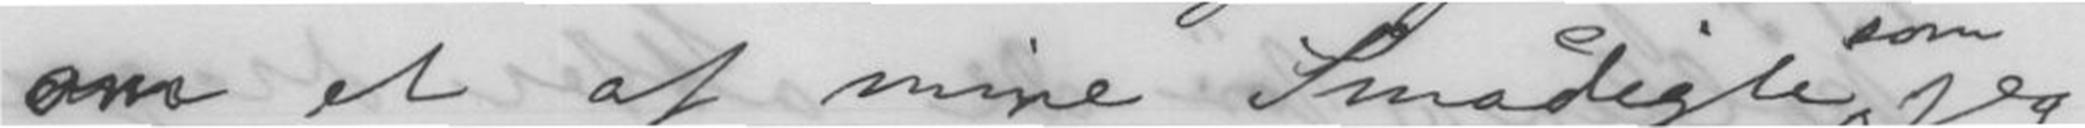om et af mine Smådigte som jeg
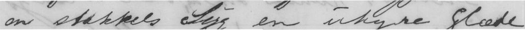en stakkels Syg en uhyre Glæde.
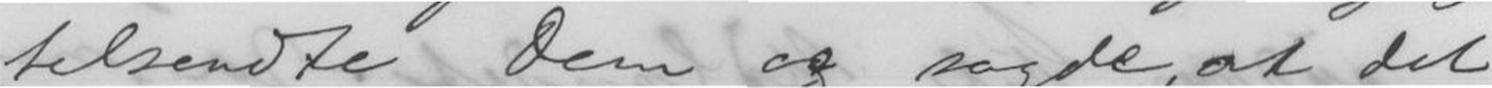tilsendte Dem og sagde, at det
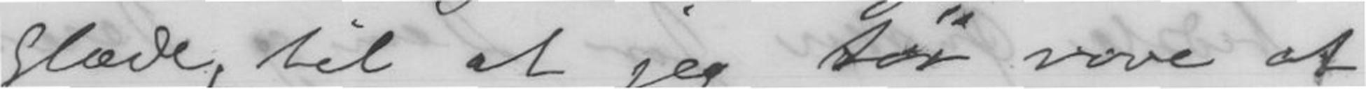Glæde, til at jeg tør vove at
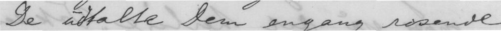- De udtalte Dem engang rosende
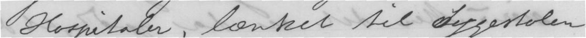Hospitaler, lænket til Sygestolen
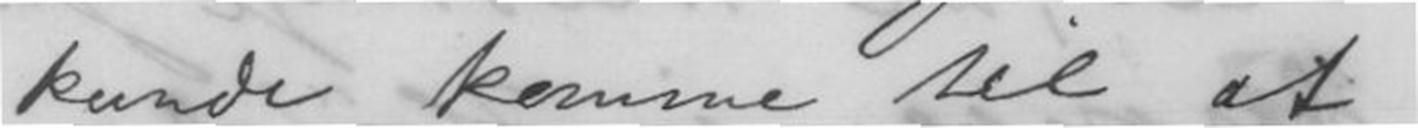kunde komme til at
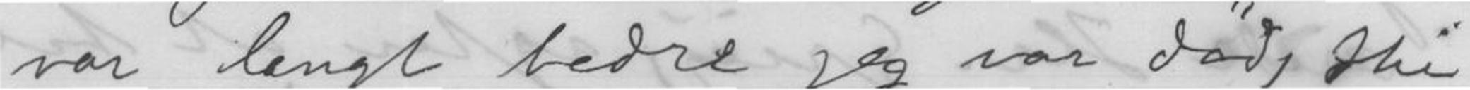var langt bedre jeg var død, thi
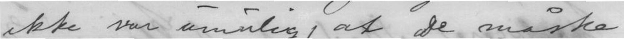ikke var umulig, at De måske
